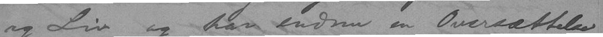og Liv og har endnu en Oversættelse
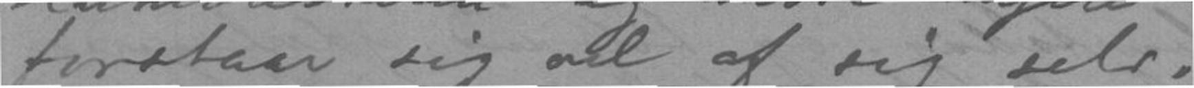forstaar sig vel af sig selv.
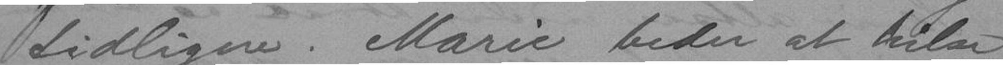tidligere. Marie beder at hilse
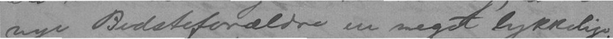nye Bedsteforældre ere meget lykkelige.
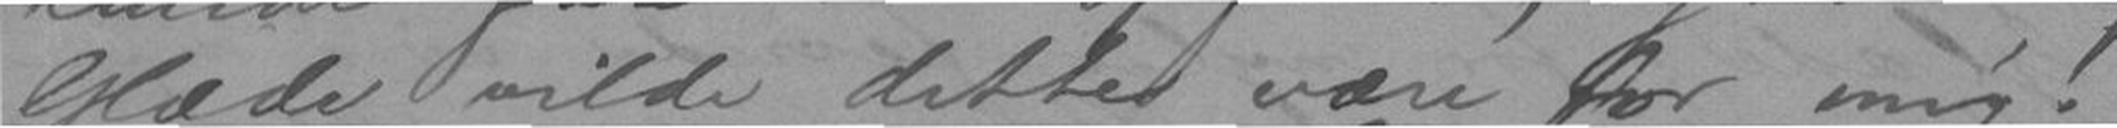Glæde vilde dette være for mig!
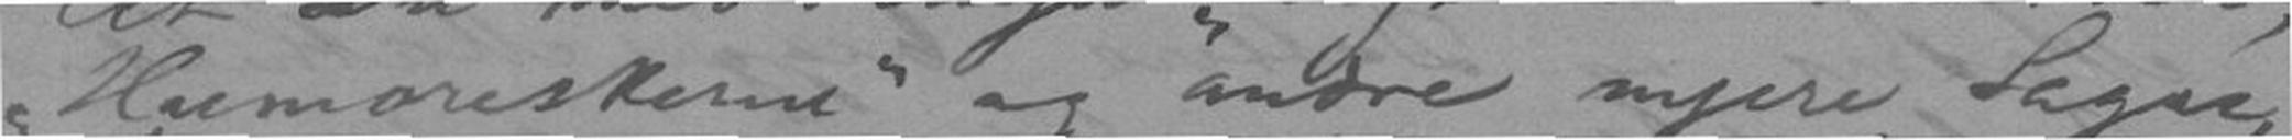Husmoreskerne og andre nyere Sager,
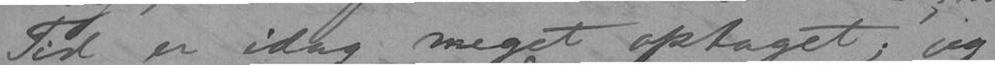Tid er idag meget optaget; jeg
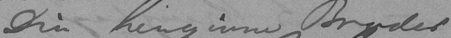Din hengivne Broder
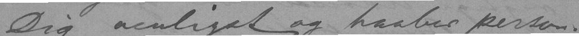Dig venligst og haaber person-
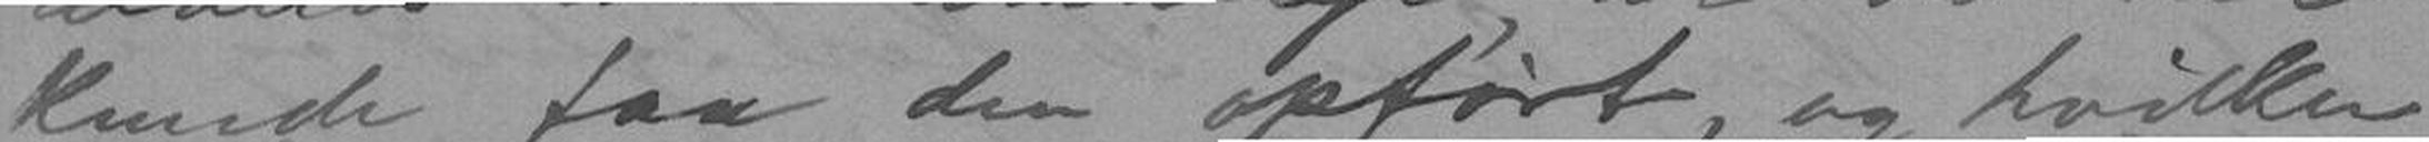kunde faa den opført, og hvilken
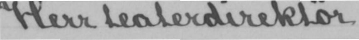Herr teaterdirektør
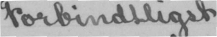Forbindtligst
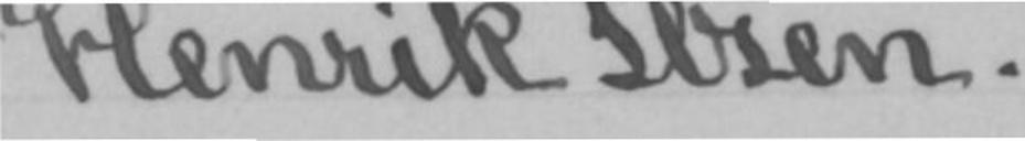Henrik Ibsen.
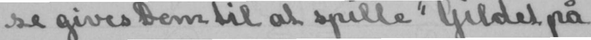se gives Dem til at spille «Gildet på
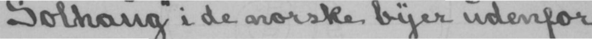Solhaug» i de norske byer udenfor
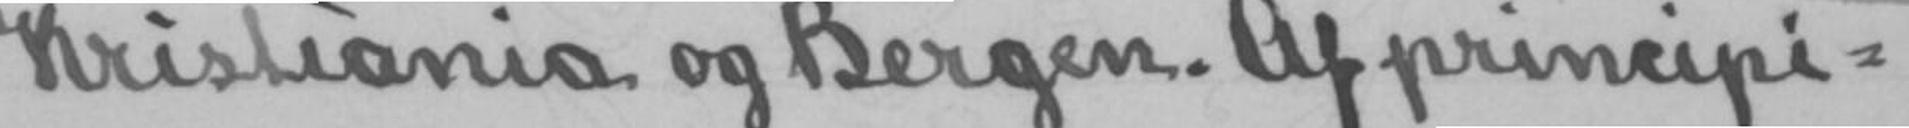Kristiania og Bergen. Af principi-
